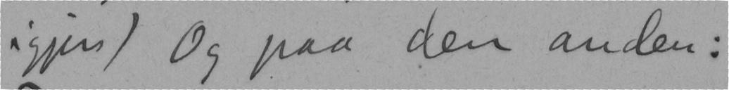igjen) Og paa den anden:
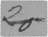20
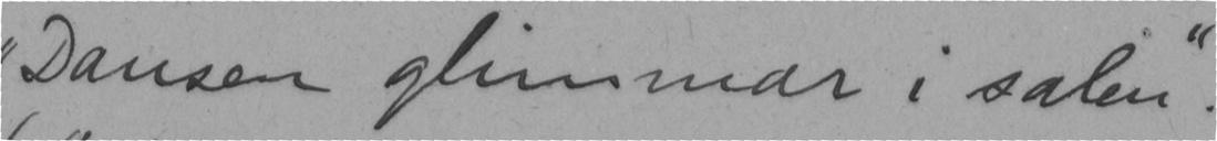"Dansen glimmar i salen".
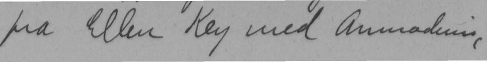fra Ellen Key med Anmodning
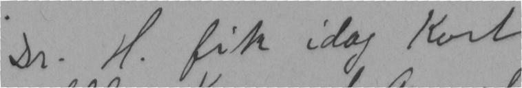Dr. H. fik idag Kort
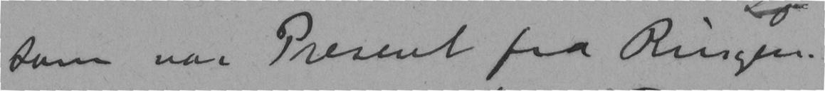som var Present fra Ringen.
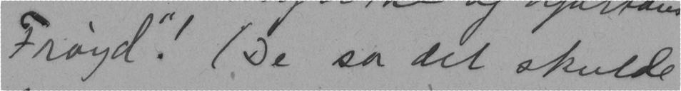Frøyd"! (De sa det skulde
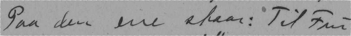Paa den ene staar: Til Fru
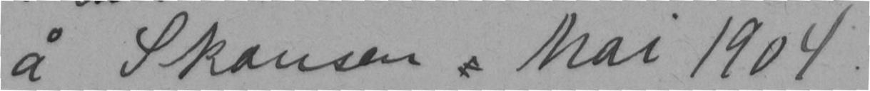å Skansen  Mai 1904.
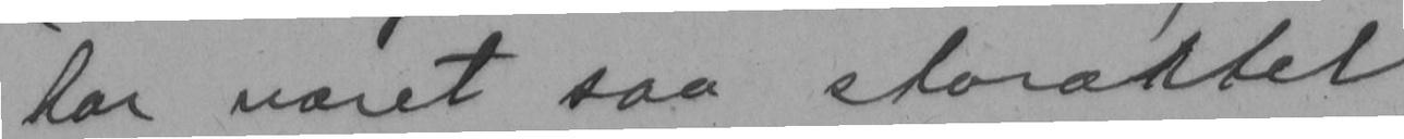har været saa storaktet
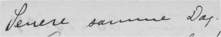Senere samme Dag.
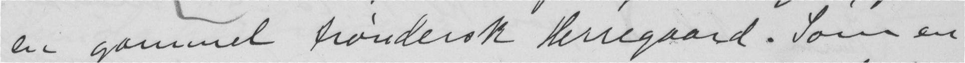en gammel trøndersk Herregaard. Som en
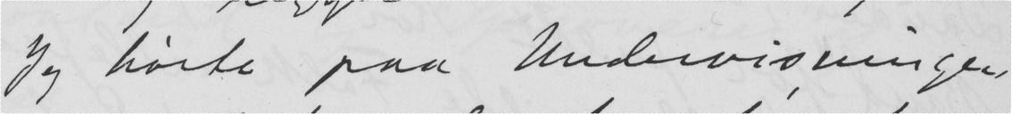Jeg hørte paa Undervisningen
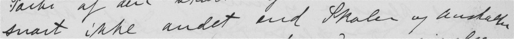snart ikke andet end Skoler og Anstalter
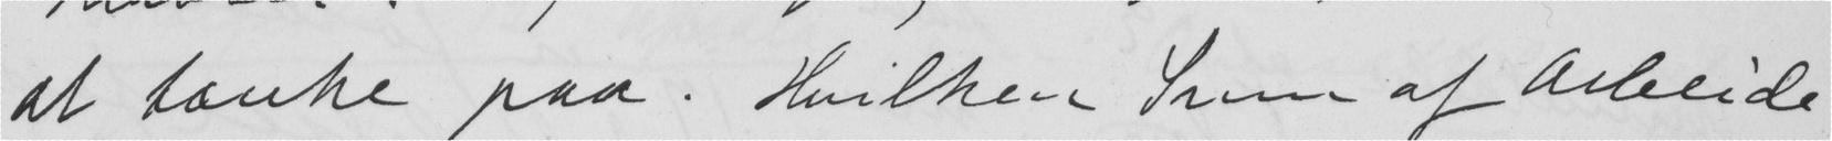at tænke paa. Hvilken Sum af arleeide
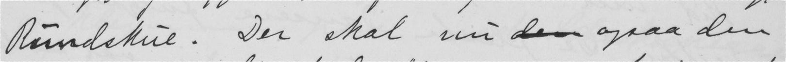Rundskue. Der skal nu  ogsaa den
